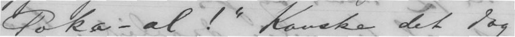Poka-al!" Kanske det dog
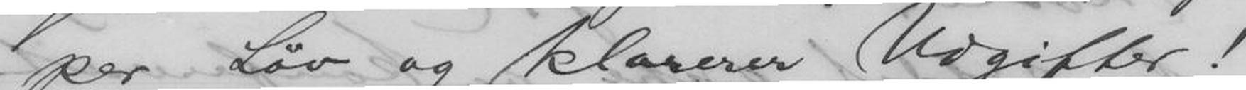per Løv og klarer Udgifter!
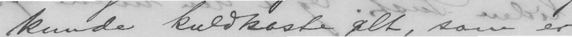kunde kulkaste alt, som er
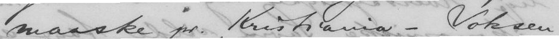maaske pr. Kristiania - Voksen-
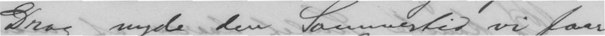Drag nyde den Sommertid, vi faar
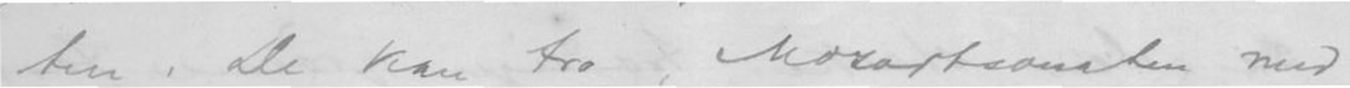ten. De kan tro, Mozartsonaten med
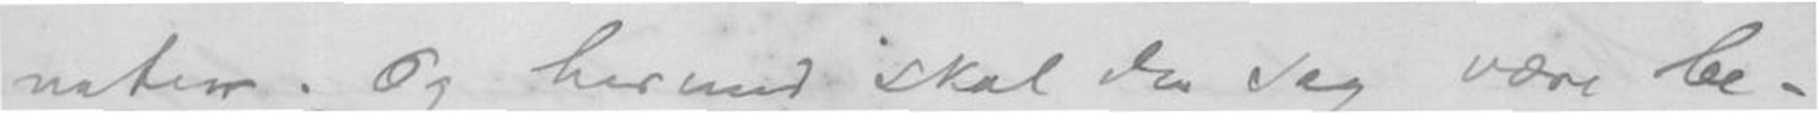natur. Og hermed skal den sag være be-
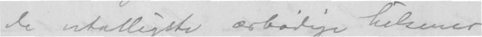de utalligste ærbødige hilsener,
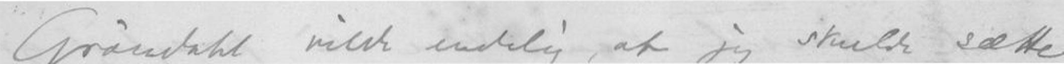Grøndahl vilde endelig, at jeg skulde sætte
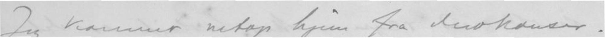Jeg kommer netop hjem fra duokonser-
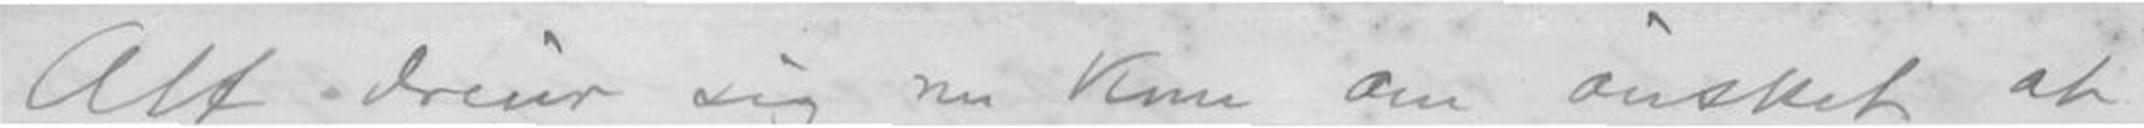Alt dreier sig nu kun om ønsket at
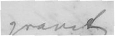gravet.
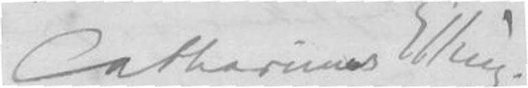Catharinus Elling.
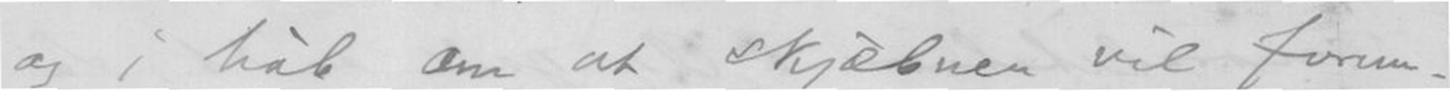og i håb om at skjæbnen vil forun-
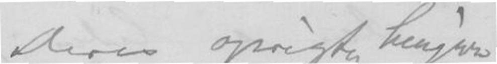Deres oprigtig hengivne
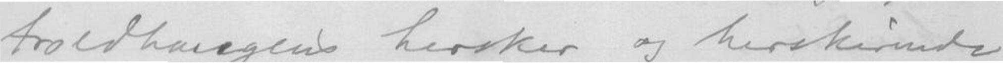troldhaugens hersker og herskerinde
